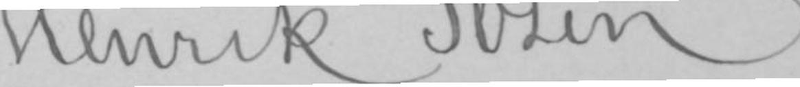Henrik Ibsen.
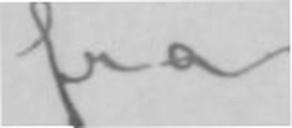fra
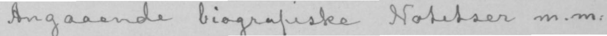Angaaende biografiske Notitser m.m.
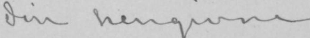din hengivne
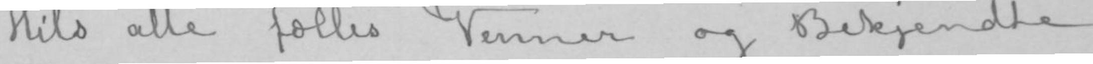Hils alle fælles Venner og Bekjendte
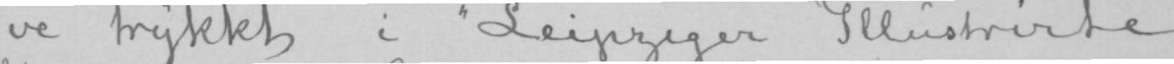ve trykket i «Leipziger Illustrirte
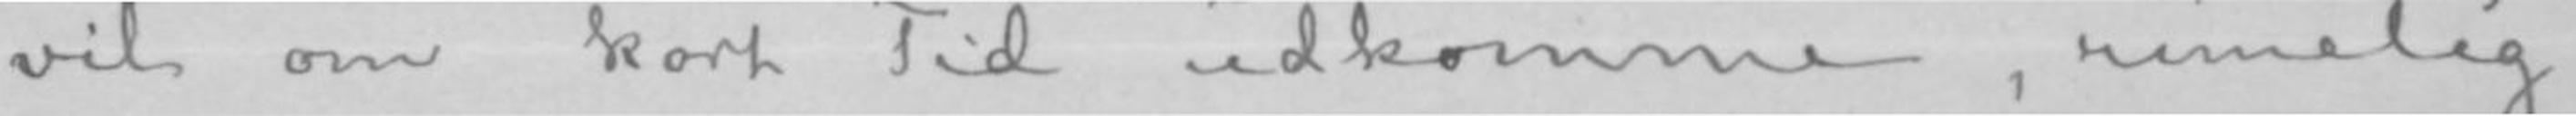vil om kort Tid udkomme, rimelig-
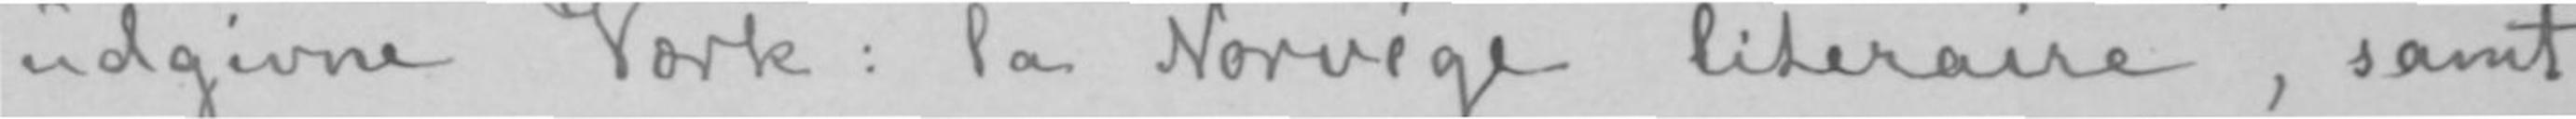udgivne Værk: la Norvége literaire», samt
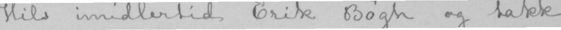Hils imidlertid Erik Bøgh og takk
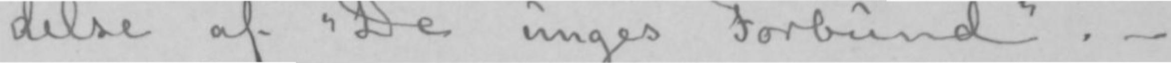delse af «De unges Forbund».-
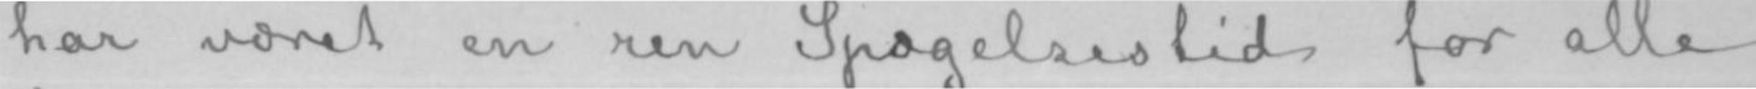har været en ren Spægelsestid for alle
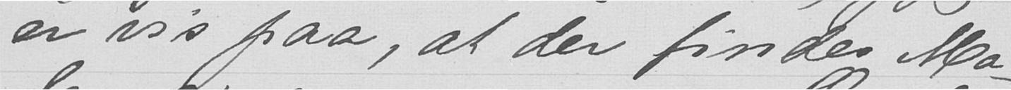er vis paa, at der findes Ma-
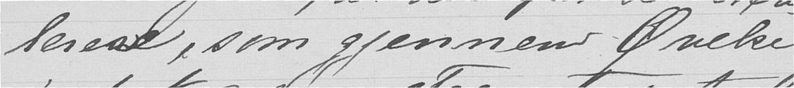lerere, som gjennem Ønske
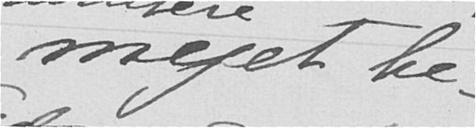meget be-
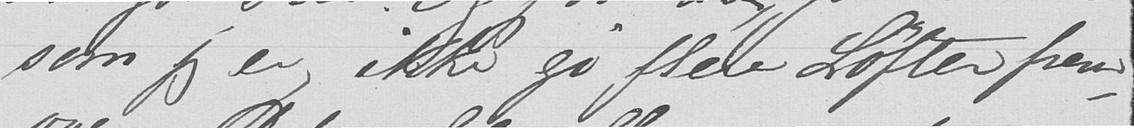som jeg er, ikke gi flere Løfter frem-
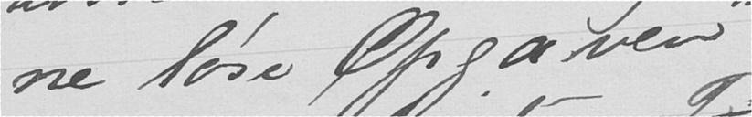ne løse Opgaven
